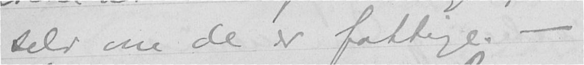selv om de er fattige. -
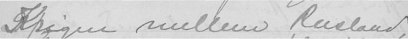Krigen mellem Rusland,
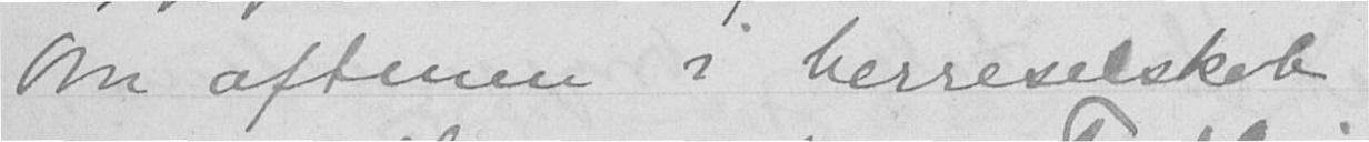Om aftenen i herreselskab
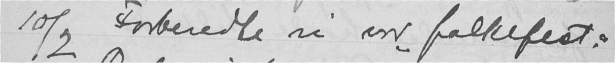10/2 Forberedte vi vor folkefest.
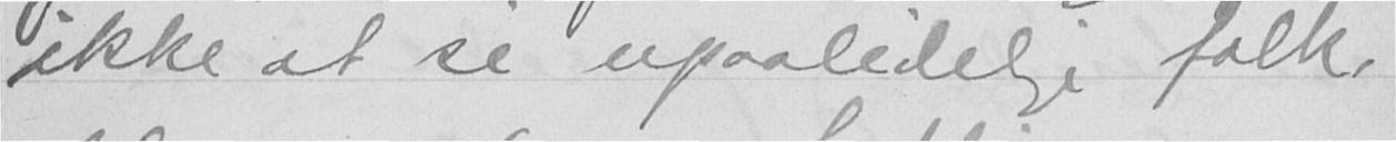ikke at si upaalidelige folk,
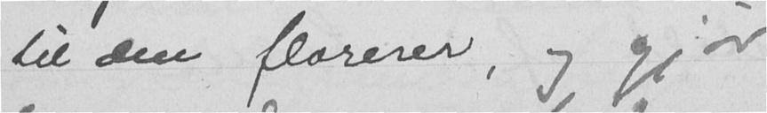til den florerer, og gjør
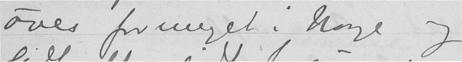øves formeget i Norge og
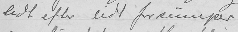lidt efter lidt forsumper
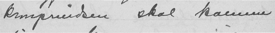kronprindsen skal komme
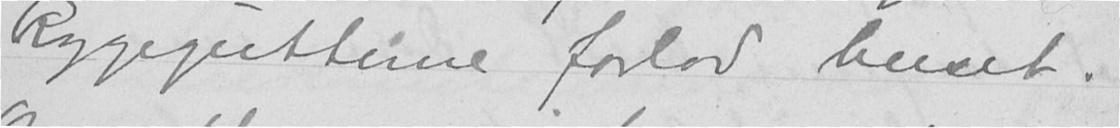Roggegutterne forlod huset.
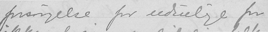forsørgelse for uduelige for
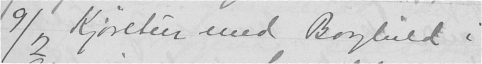9/2 Kjøretur med Borghild i
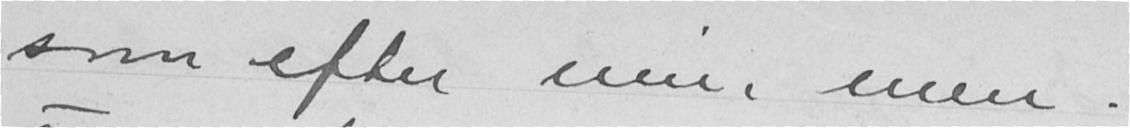som efter min men.
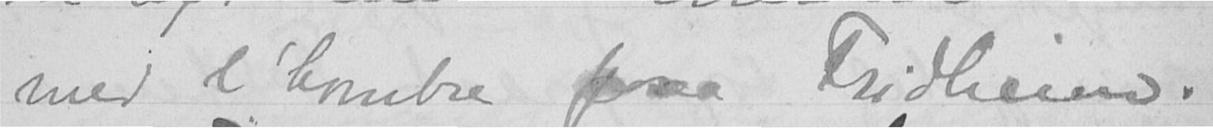med l'hombre paa Fridheim.
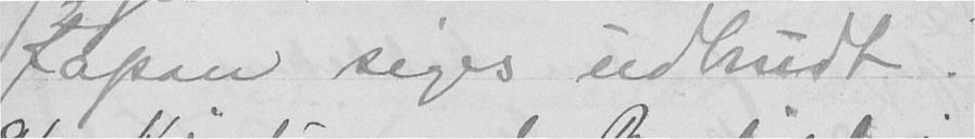Japan siges udbrudt.
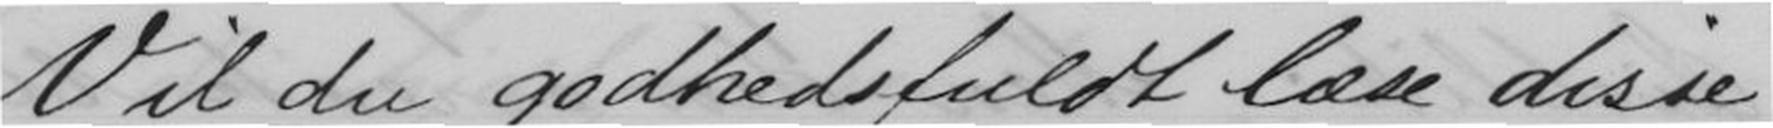Vil du godhedsfuldt læse disse
